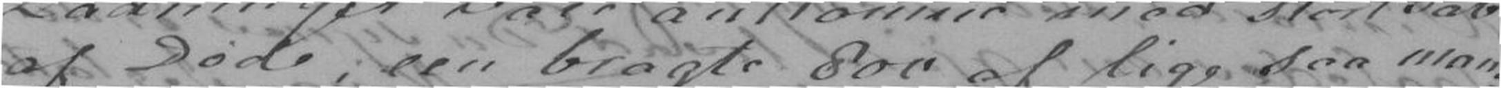af døde; een bragte 800 af ligesaa man-
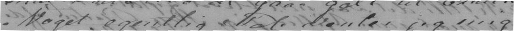Noget egentlig Tab venter jeg mig
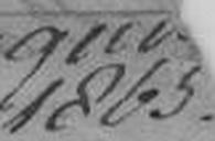1865
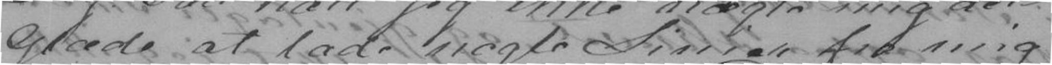Glæde at lade nogle Linier fra mig
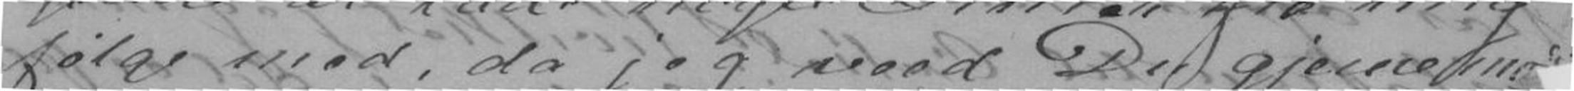følge med, da jeg veed Du gjærne mod-
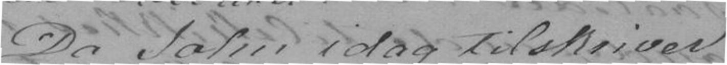Da John idag tilskriver
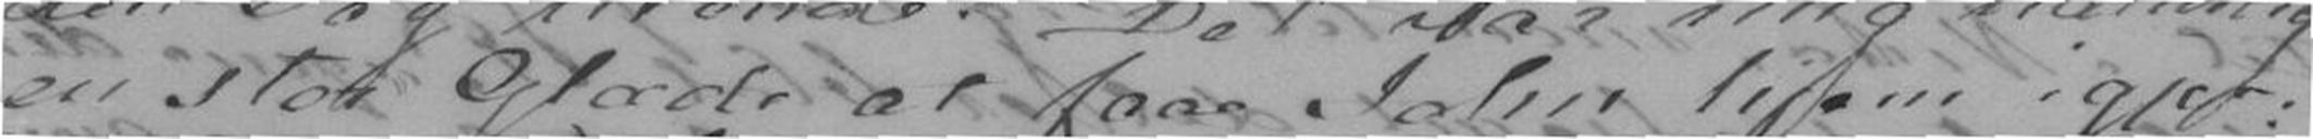en stor Glæde at faae John hjem igjen,
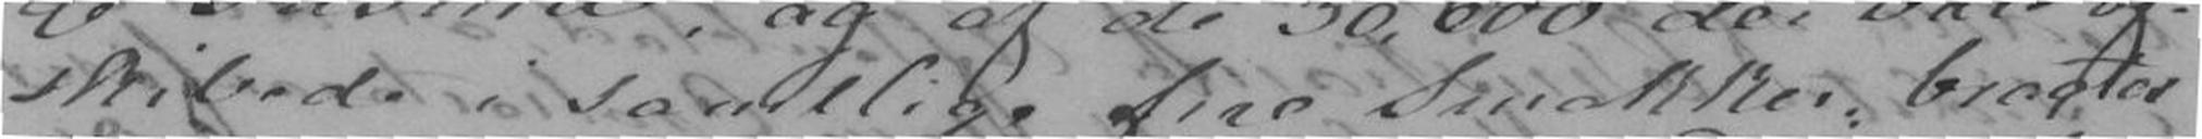skibede i Samtlige fire Smakker, bragtes
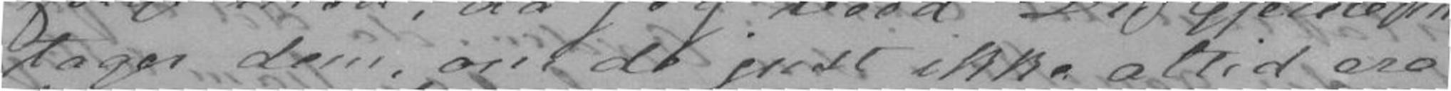tager dem om de just ikke altid ere
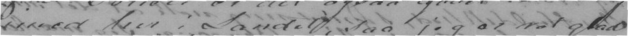imod her i Landet, saa jeg er ret glad
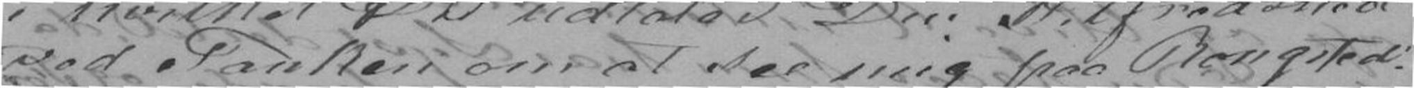ved Tanken om at see mig paa Rongsted!
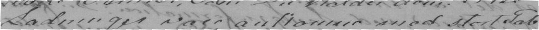Ladninger vare andkommen med stort Tab
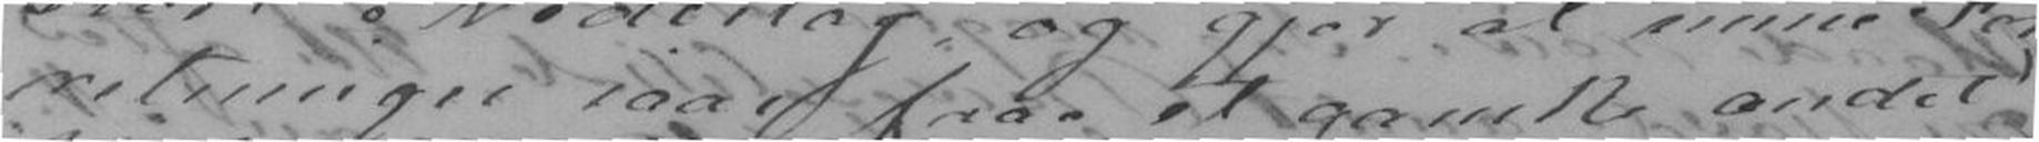retninger iaar faae et ganske andet
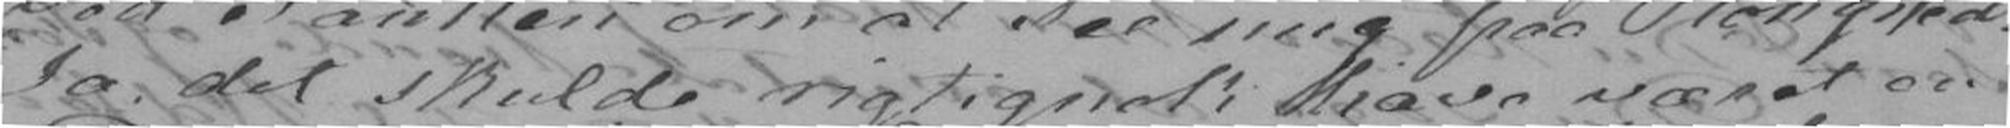Ja, det skulde rigtignok have været en
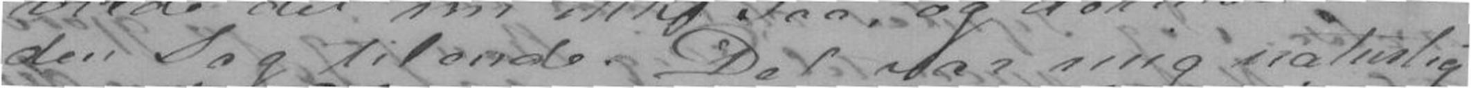den Sag tilende. Det var mig naturlig
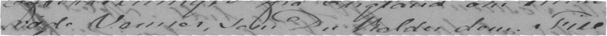røde Venner, som Du kalder dem. Fire
